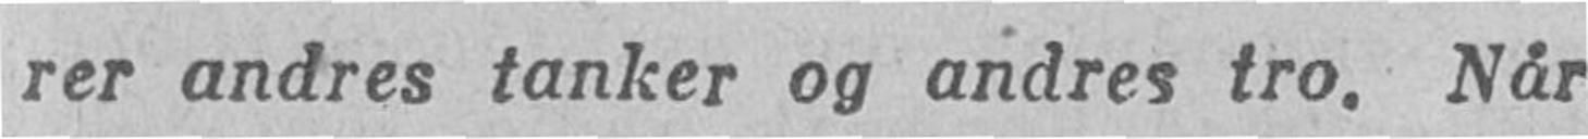rer andres tanker og andres tro. Når
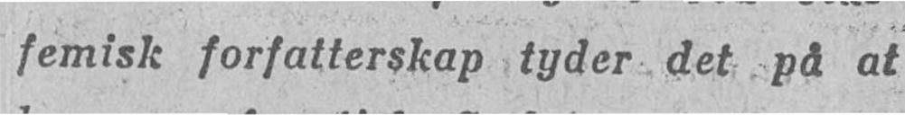femisk forfatterskap tyder det på at
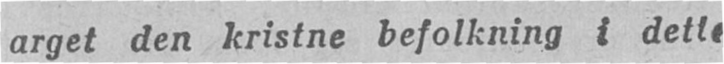arget den kristne befolkning i dette
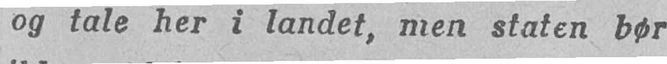og tale her i landet, men staten bør
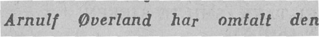Arnulf Øverland har omtalt den
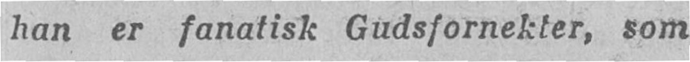han er fanatisk Gudsfornekter, som
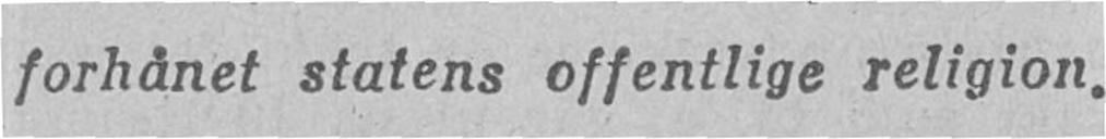forhånet statens offentlige religion.
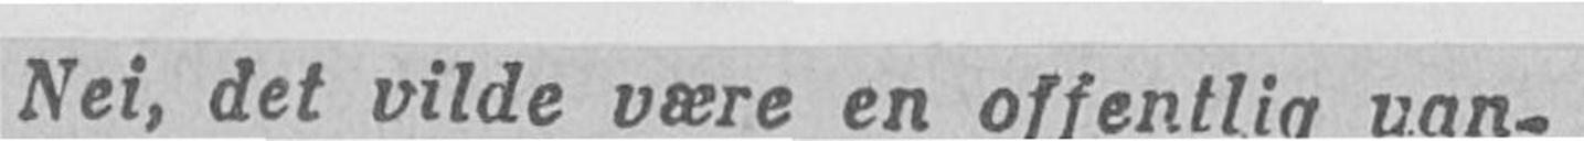Nei, det vilde være en offentlig uan-
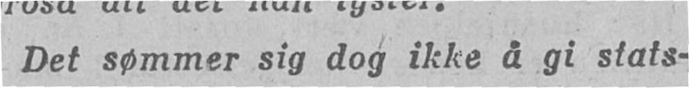Det sommer sig dog ikke å gi stats-
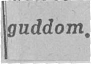guddom.
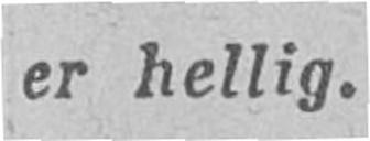er hellig.
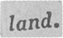land.
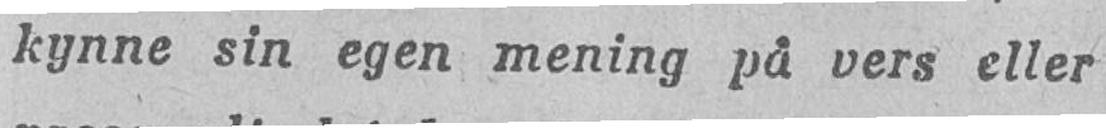kynne sin egen mening på vers eller
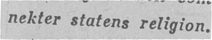nekter statens religion.
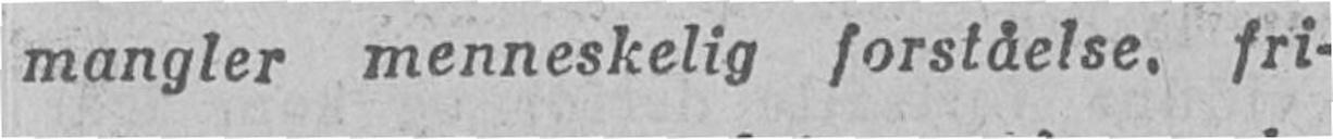mangler menneskelig forståelse, fri-
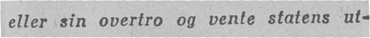eller sin overtro og vente statens ut-
